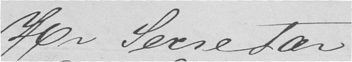Hr Secretær
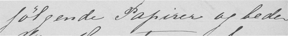følgende Papirer og beder
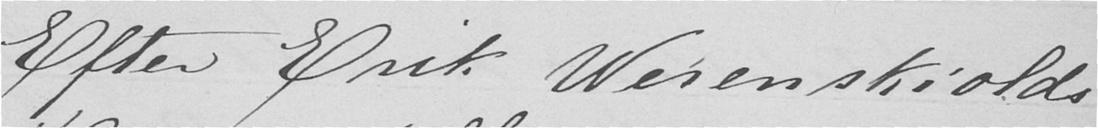Efter Erik Werenskiolds
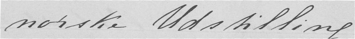norske Udstilling
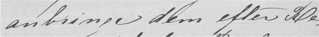anbringe dem efter Re-
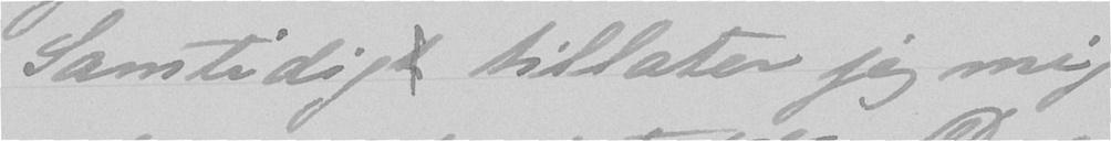Samtidigt tillater jeg mig
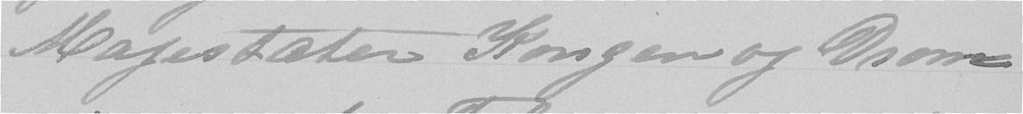Majestæter Kongen og Dron-
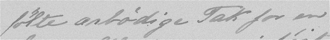følte ærbødige Tak for en
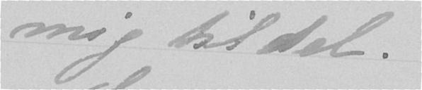mig til del.
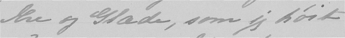Ære og Glæde, som jeg høit
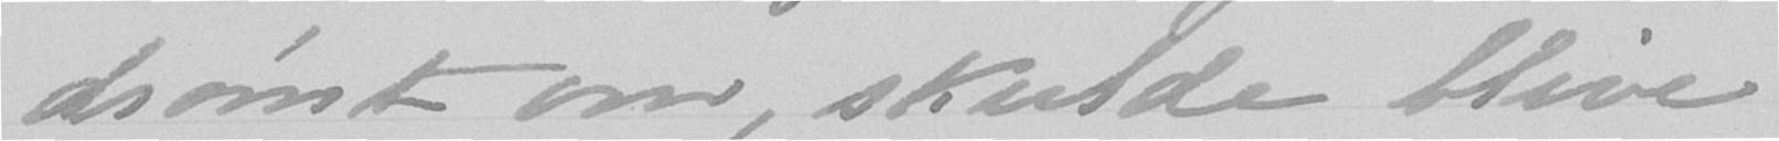drømt om, skulde blive
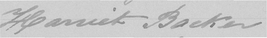Harriet Backer
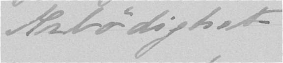Ærbødighet
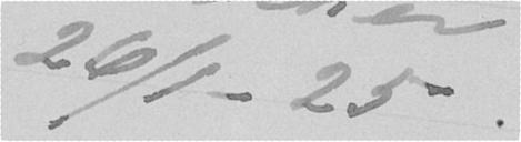26/1 - 25.
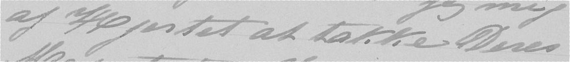af Hjertet at takke Deres
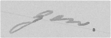gen.
